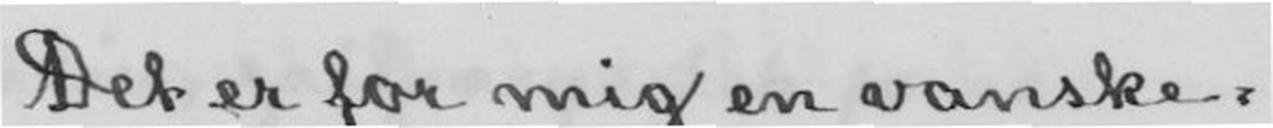Det er for mig en vanske-
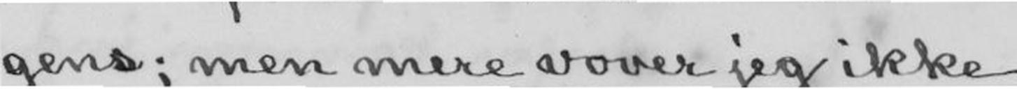gens; men mere vover jeg ikke
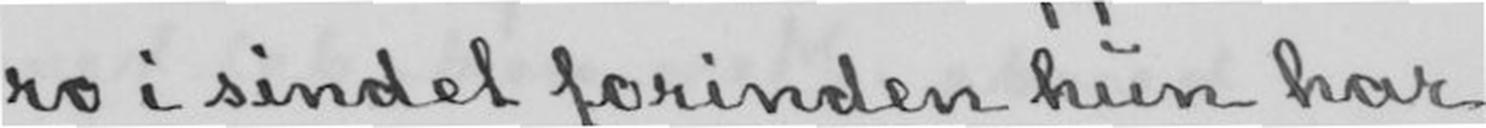ro i sindet forinden hun har
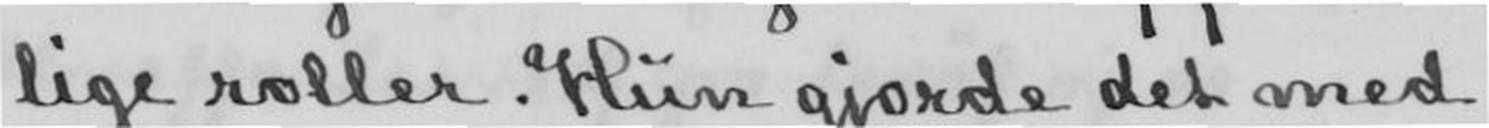lige roller. Hun gjorde det med
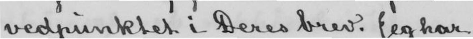vedpunktet i Deres brev. Jeg har
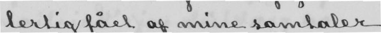lertig fået af mine samtaler
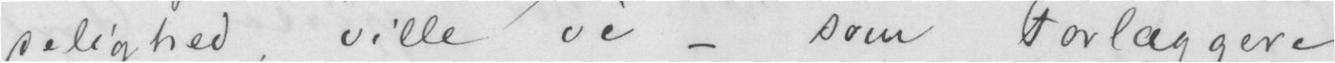delighed, ville vi - som Forlæggere
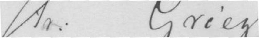Hr. Grieg
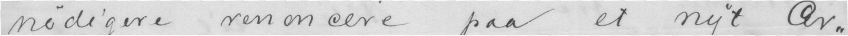nødigere renoncere paa et nyt Ar-
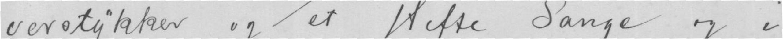verstykke og et Hefte Sange og i
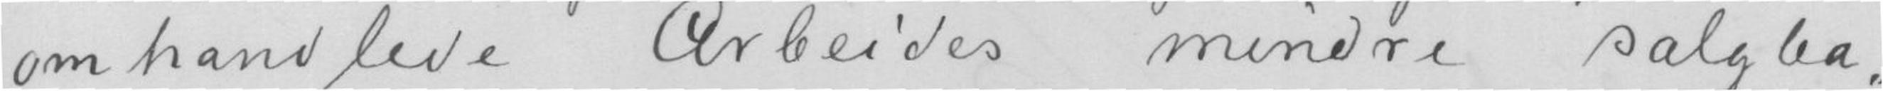om hand leve Arbeides mindre salgba-
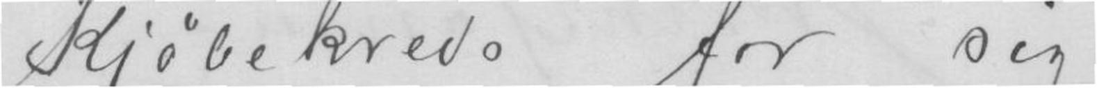Kjøbekreds for sig.
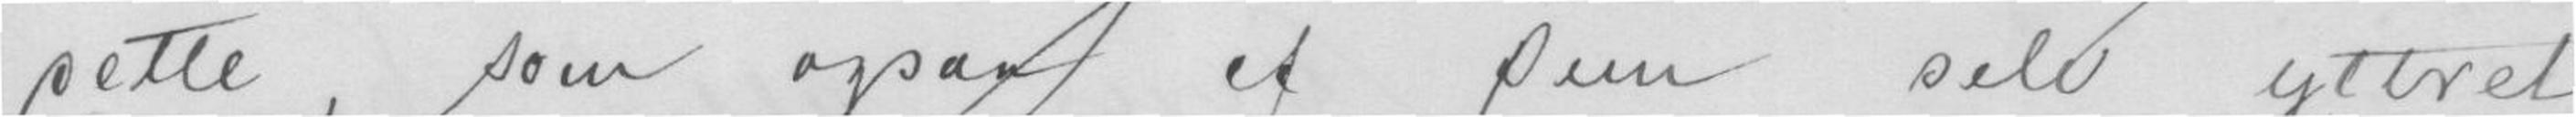sette, som ogsaa af Den selv yttrel,
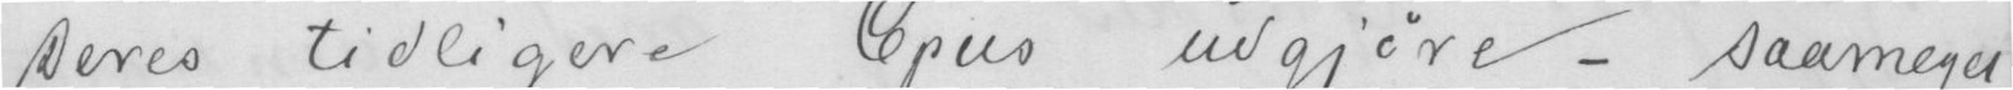Deres tidligere Opus udgjøre - saameget
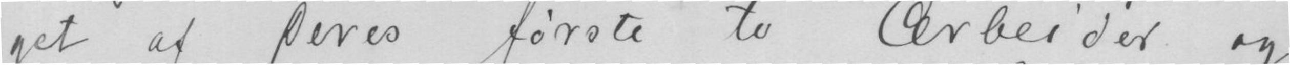get af Deres første to Arbeider og
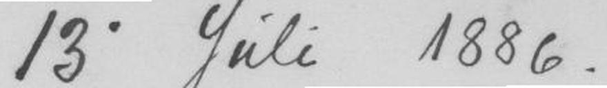13 Juli 1886
